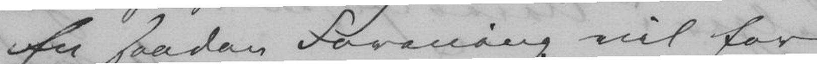En saadan Forening vil for
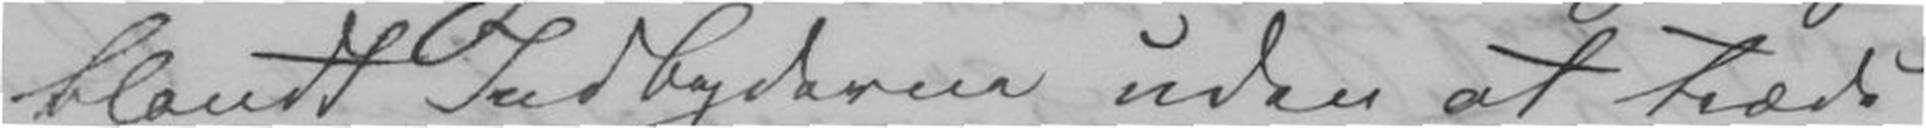blandt Indbyderne uden at træde
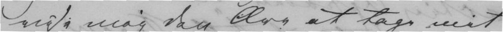vise mig den Ære at tage mit
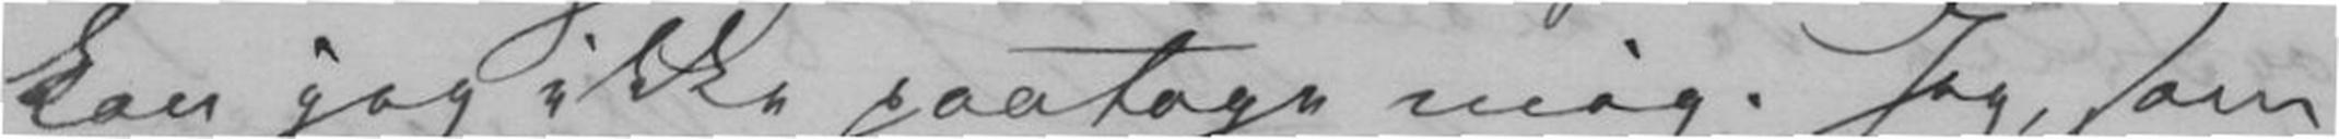kan jeg ikke paatage mig. Jeg, som
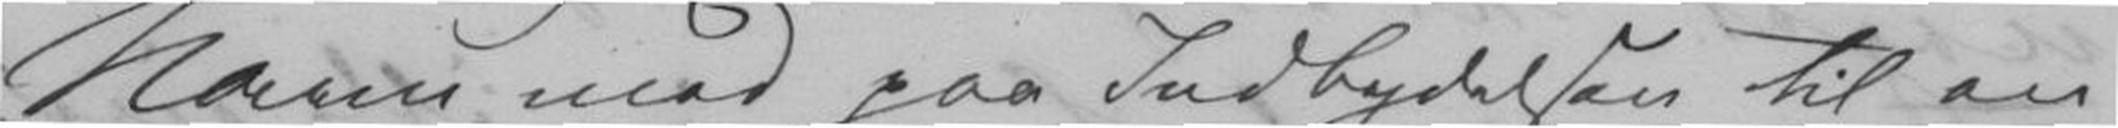Navn med paa Indbydelsen til en
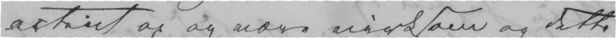activt op og være virksom og ditto
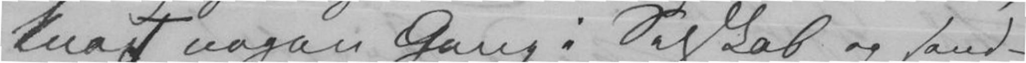knapt nogen Gang i selskab og sand-
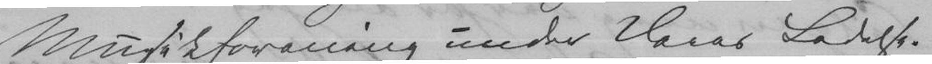Musikforening under Deres Ledelse.
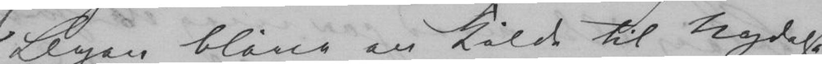Leyers blive en Kilde til Nydelse
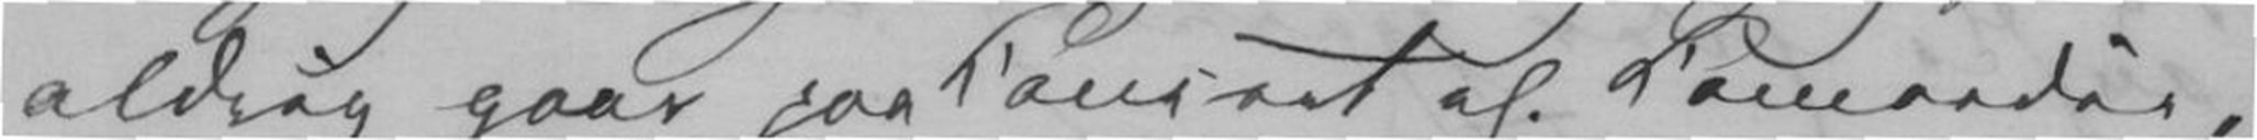aldrig gaar paa Consert af Lamaadis,
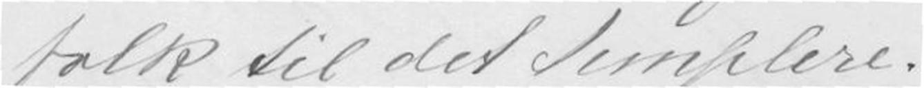folk til det Simplere.-
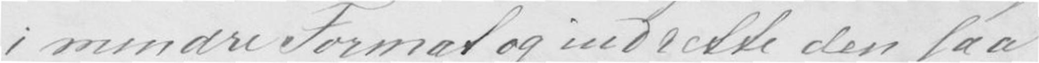i mindre Format og indrette den faar
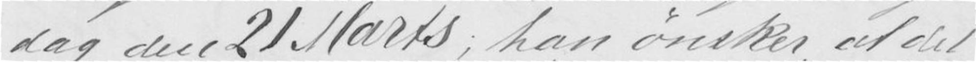dag den 21 Marts, han ønsker at det
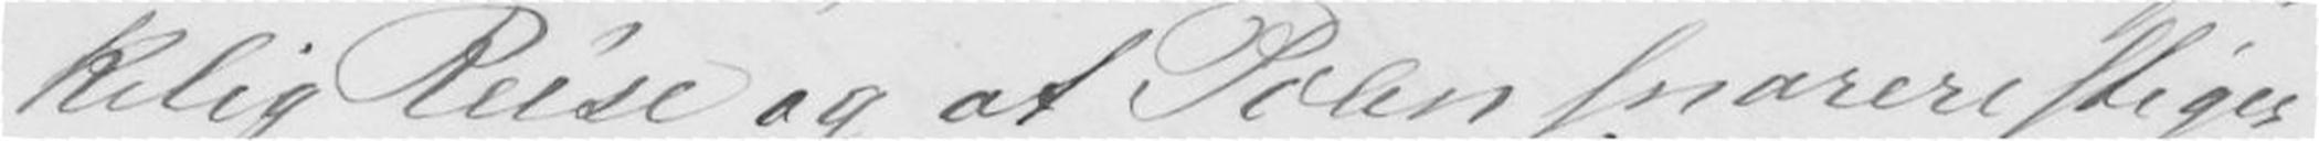kelig Reise og at Polen snarere
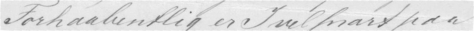Forhaabentlig er i vel snart paa
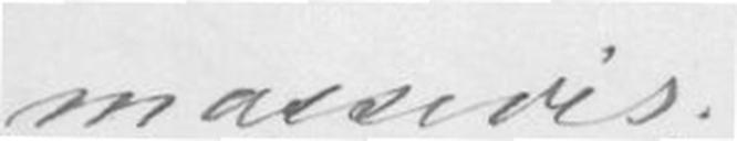massevis.
